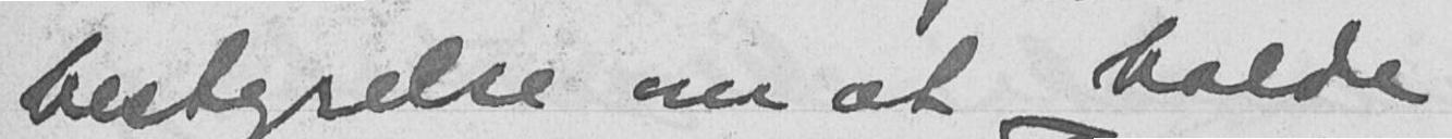bestyrelse om at holde
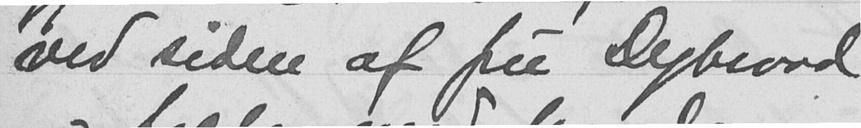ved siden af fru Dybwad
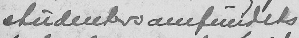studentersamfundets
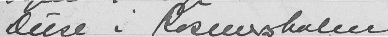Duse i Rosmersholm
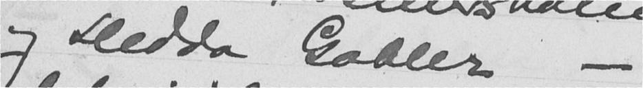og Hedda Gabler -
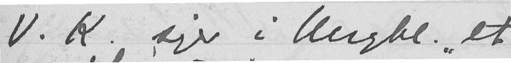V. K. siger i Mrgbl. "et
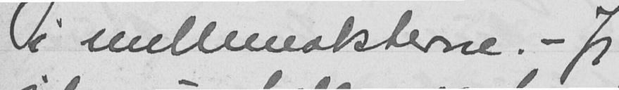i mellem akterne. - Jeg
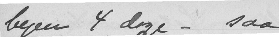byen 4 dage - saa
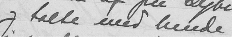og talte med hende
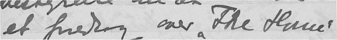et foredrag over "The Home"
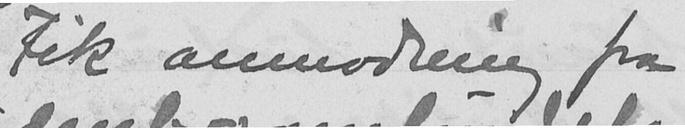Fik anmodning fra
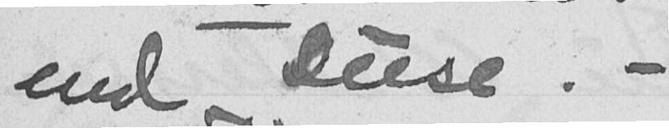end Duse. -
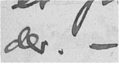der. -
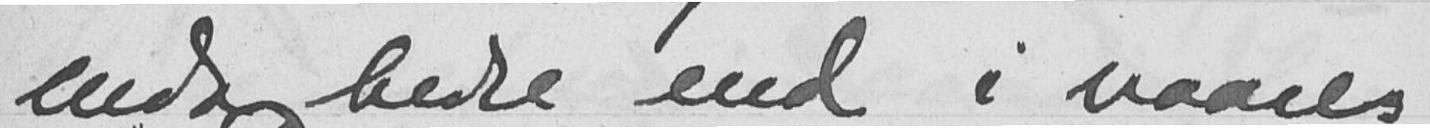enda bedre end i vaares
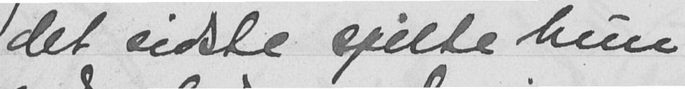det sidste spilte hun
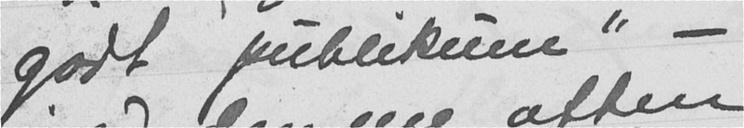godt publikum” -
